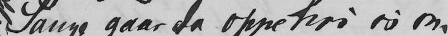Sange gaar da oppe hos os om
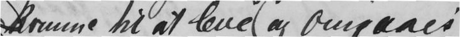komme til at leve og Omgaaes
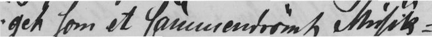get som et sammendrømt Musik-
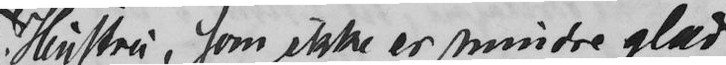Hustru, som ikke er mindre glad
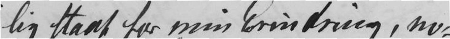lig staat for min Erindring, no-
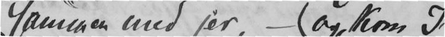sammen med jer, - og, kom I
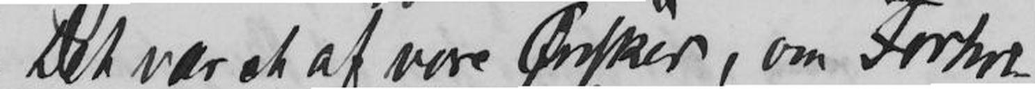Det var et af vore Ønsker, om Forhol-
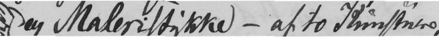og Maleristykke - af to Kunstnere,
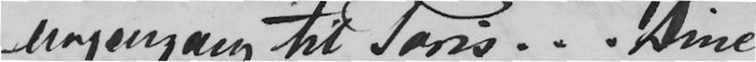nog engang til Paris... Dine
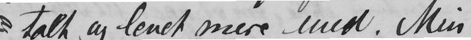talt og levet mere med. Min
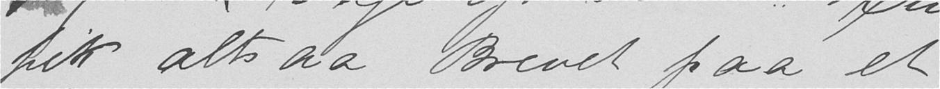fik altsaa Brevet paa et
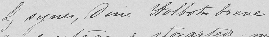Jeg synes, Dine Kolbotnbreve
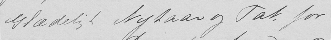Glædeligt Nytaar og Tak for
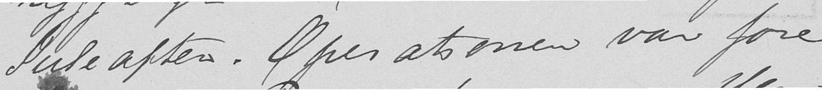Juleaften. Operationen var fore-
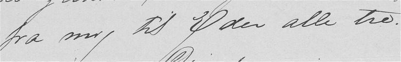fra mig til Eder alle tre.
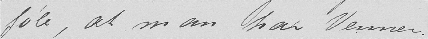føle, at man har Venner!
